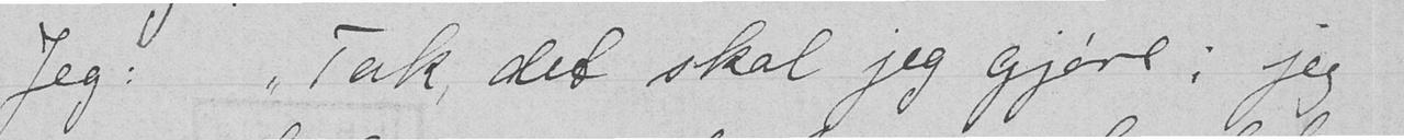Jeg: Tak, det skal jeg gjøre; jeg
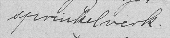sprinkelverk.
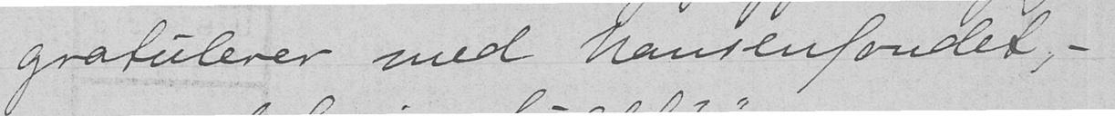gratulerer med Nansenfondet, -
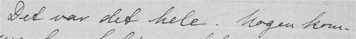Det var det hele. Nogen kom-
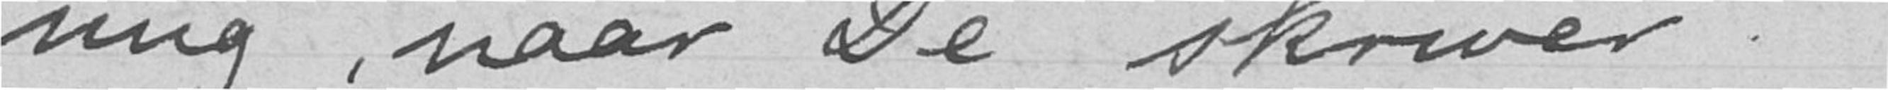mig, naar De skriver."
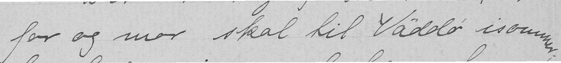far og mor skal til Väddø isommer;
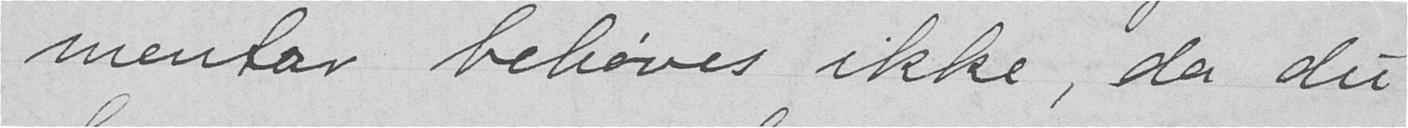mentar behøves ikke, da du
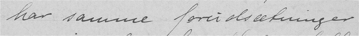har samme forudsætninger
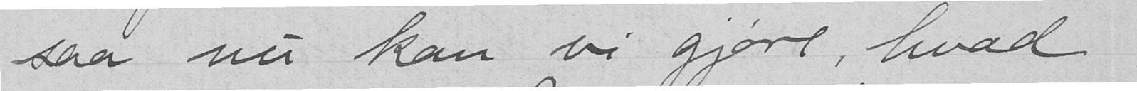saa nu kan vi gjøre, hvad
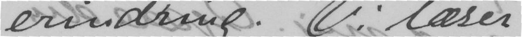erindring. Vi læser
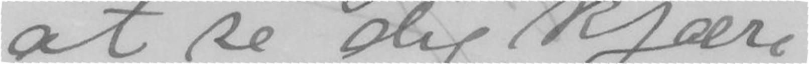at se dig kjære
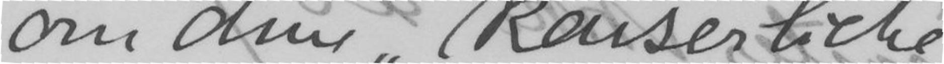om den Raiserliche
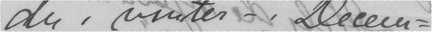du i vinter i Decem=
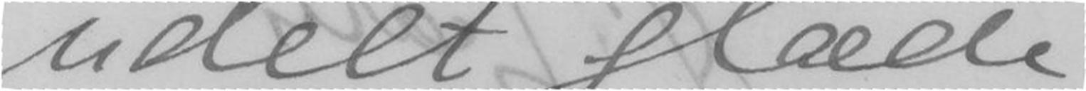udelt glæde
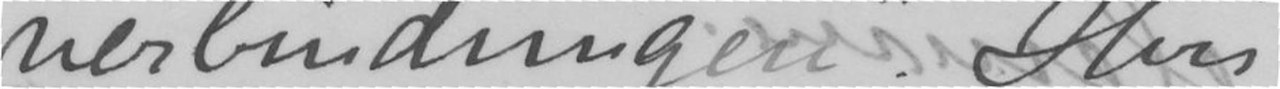verbindungen Hvis
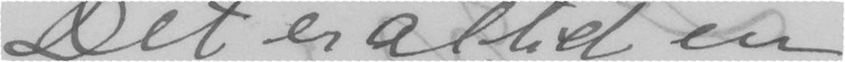Det er altid en
